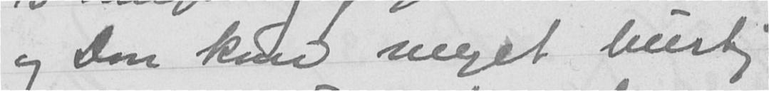og Don kom meget hurtig
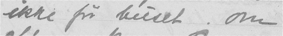ikke før huset. Om
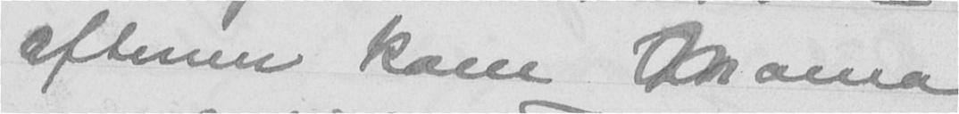aftenen kom Mama
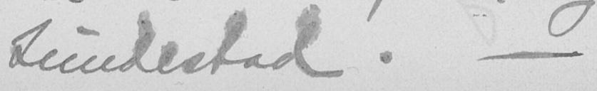Lundestad. -
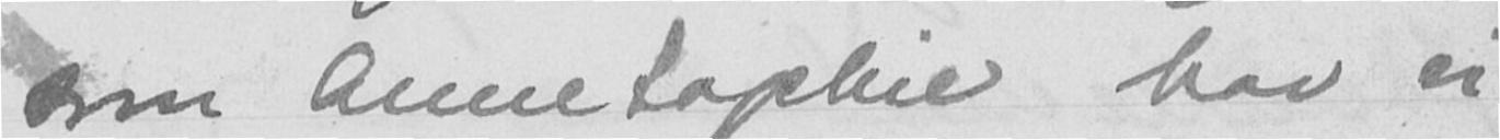som Anne Sophie har vi
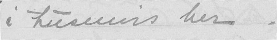i tusenvis her.
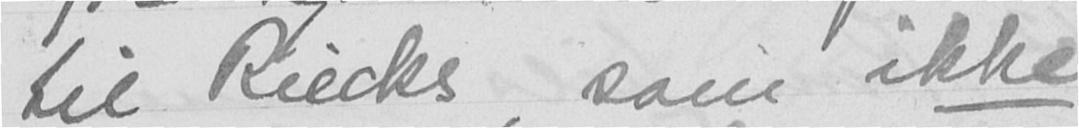til Riecks, som ikke
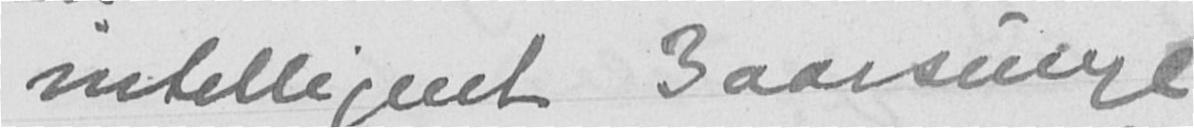intelligent 3aarsunge
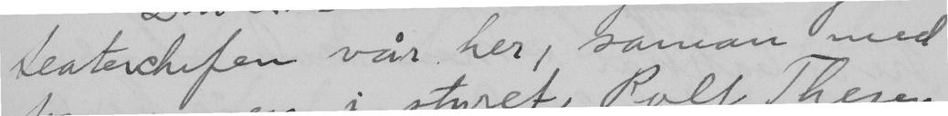teaterchefen vår her, saman med
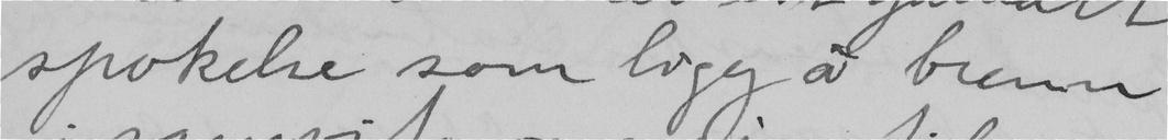spökelse som ligg å brenn
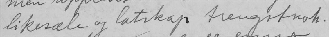likesæle og latskap trengst nok.
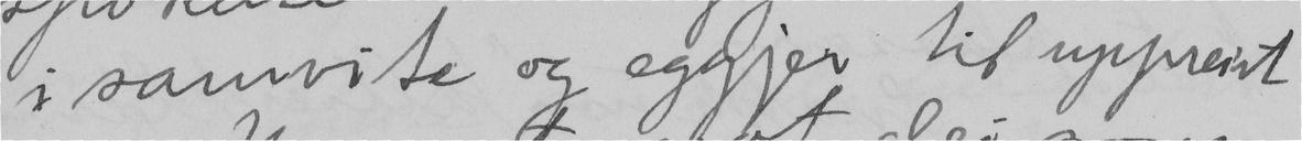i samvite og eggjer til uppreist
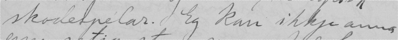skodespelar. Eg kan ikkje anna
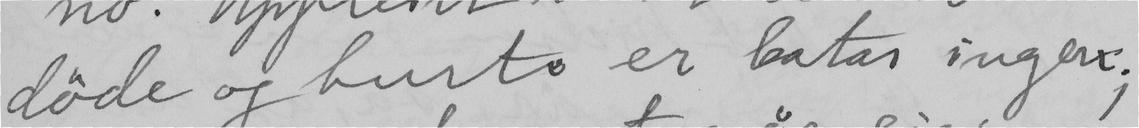döde og huste er batas ingen;
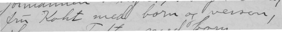fru Koht med born og verson,
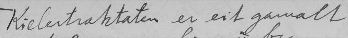Kielertraktaten er eit gamalt
